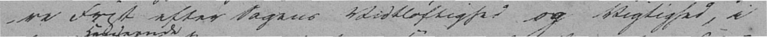re Frist efter Sagens Vidtløftighed og Vigtighed, i
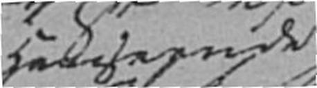Henseende
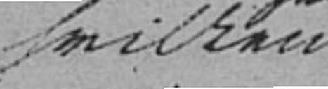hvilken
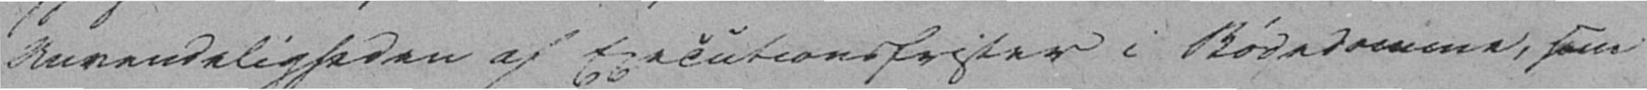Anvendeligheden af Executionsfrister i Bødedomme, som
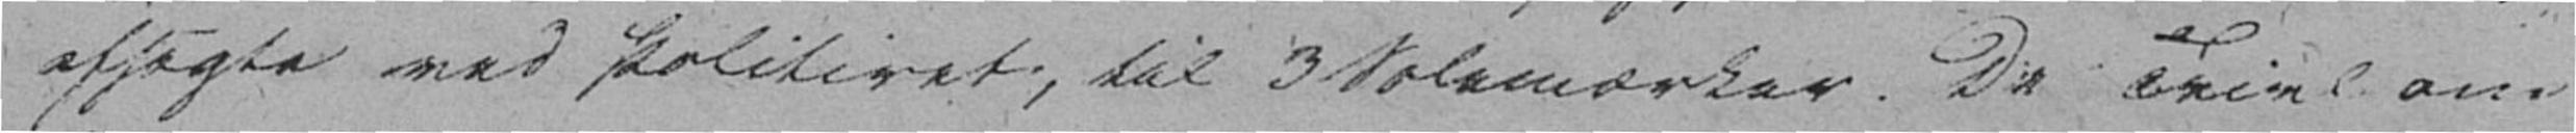afsagte ved Politiret, til 3 Solemærker. De Tvivl om
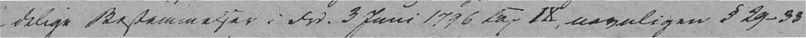delige Bestemmelser i Frd. 3 Juni 1796 Cap IX, navnligen § 29-33
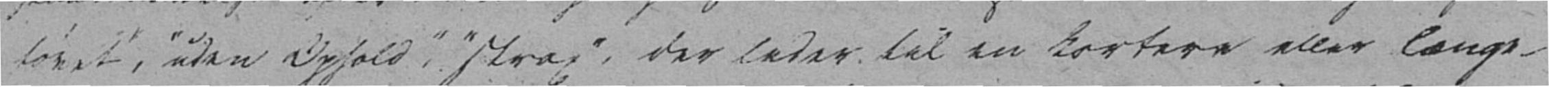tøvet", "uden Ophold", "strax", der leder til en kortere eller længe-
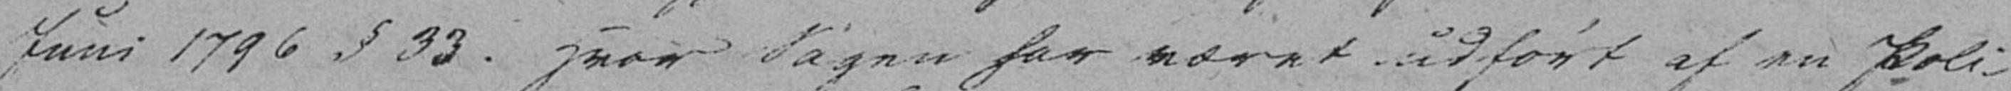Juni 1796 § 33. Hvor Sagen har været udført af en Poli-
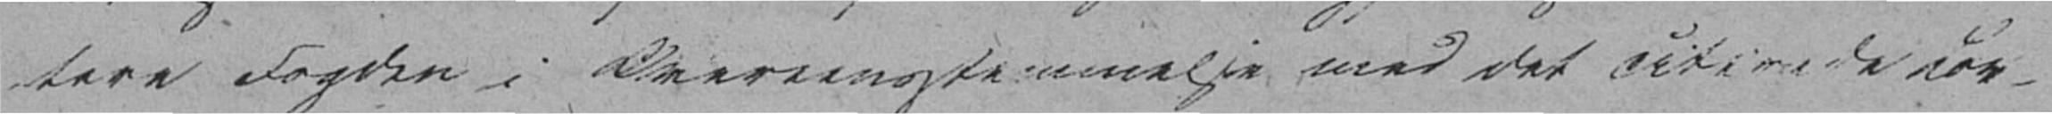tere Fogden i Overeensstemmelse med det citerede Lov-
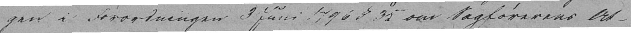gen i Forordningen 3 Juni 1796 § 35 om Sagførerens At-
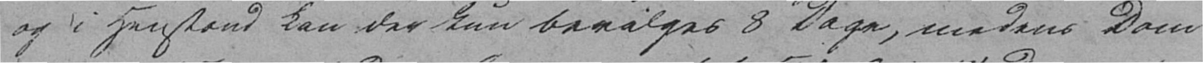og i Henstand kan der kun bevilges 8 Dage, medens Dom
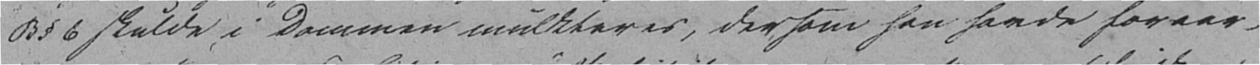B § 6 skulde i Dommen mulkteres, dersom han havde foraar-
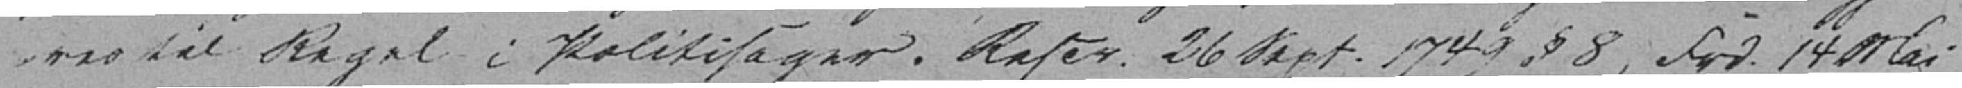res til Regel i Politisager. Rescr. 26 Sept. 1749 § 8, Frd. 14 Mai
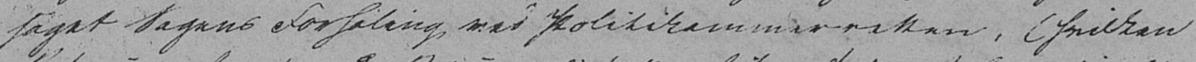saget Sagens Forhaling ved Politikammerretten, (hvilken
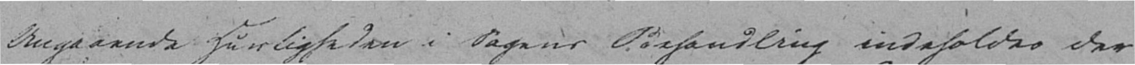Angaaende Hurtigheden i Sagens Behandling indeholdes der
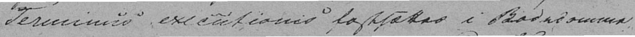Terminus executionis fastsættes i Bødedomme
